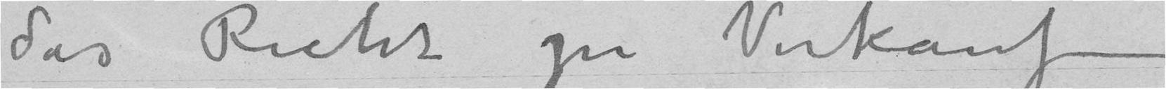das Recht zu Verkauf
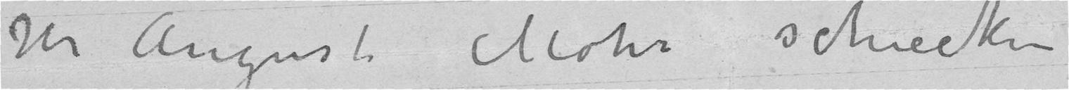Hr August Mohr schiecken
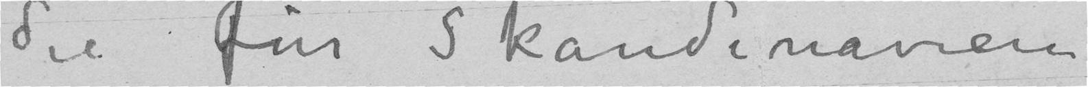die für Skandinavien
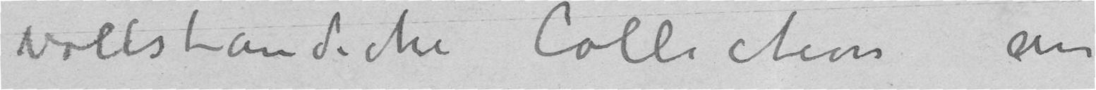vollstandiche Collection an
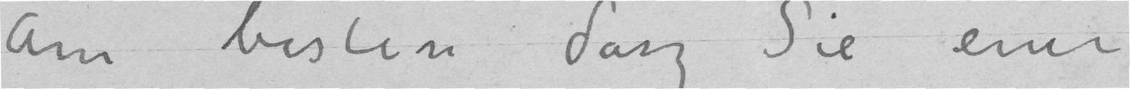am besten dasz Sie eine
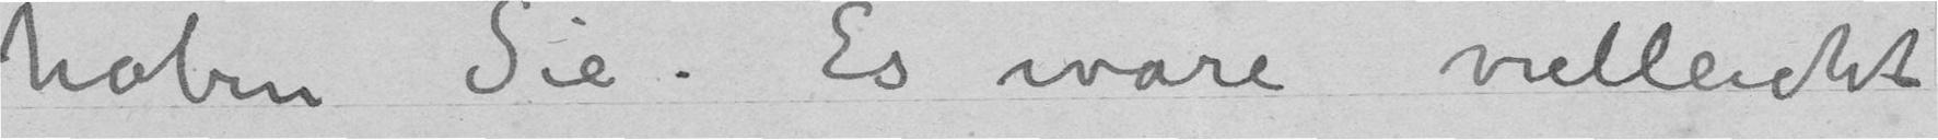haben Sie. Es ware vielleicht
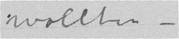wollten –
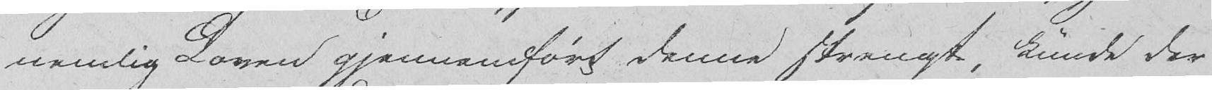nemlig Loven gjennemført denne strengt, kunde der
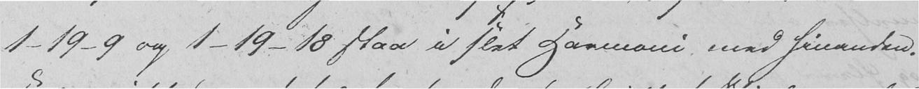1-19-9 og 1-19-18 staa i slet Harmoni med hinanden.
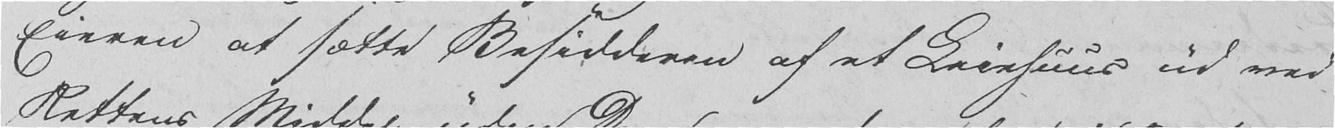Eieren at sætte Besidderen af et Leiehuus ud ved
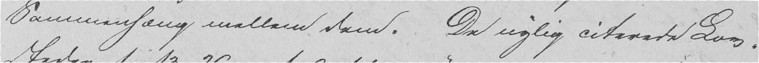Sammenhæng mellem dem. De nylig citerede Lov-
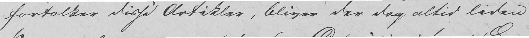fortolker disse Artikler, bliver der dog altid liden
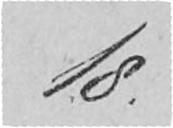18.
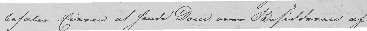befaler Eieren at hævde Dom over Besidderen af
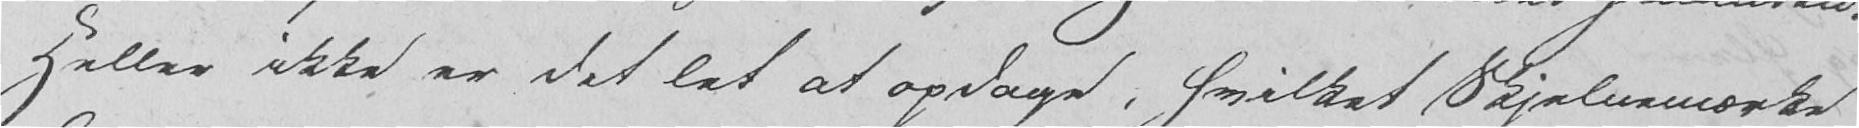Heller ikke er det let at opdage, hvilket Skjelnemærke
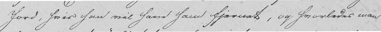Jord, hvis han vil have ham fjernet, og hvorledes man
